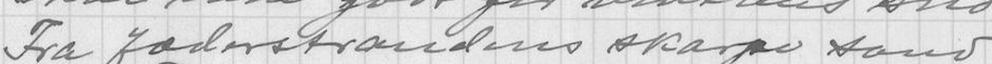Fra jæderstrandens skarpe sand
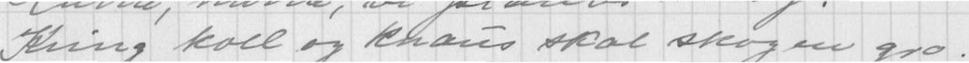Kring koll og knaus skal skogen gro.
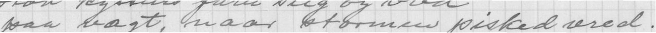paa vægt, naar stormen pisked æred.
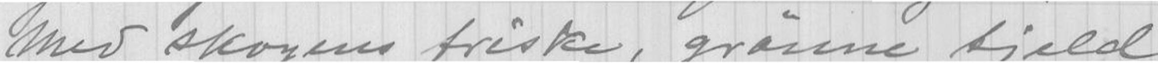Med skogens friske, grønne tjeld
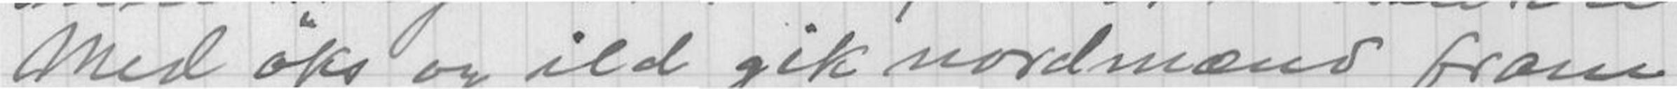Med øks og ild gik nordmænd fram
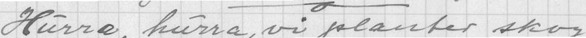Hurra, hurra, vi planter skog
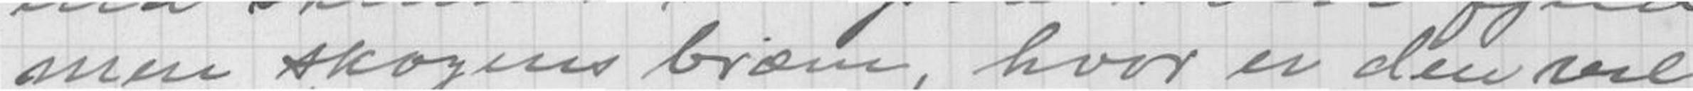mens skogens bræm, hvor er den vel
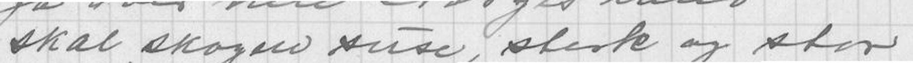skal skogen suse, sterk og stor
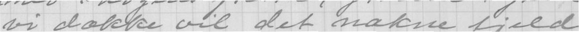vi dække vil det nakne fjeld
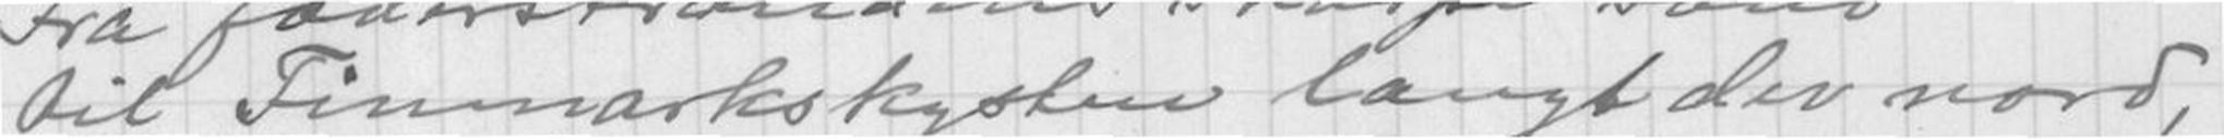til Finmarkskysten langt der nord,
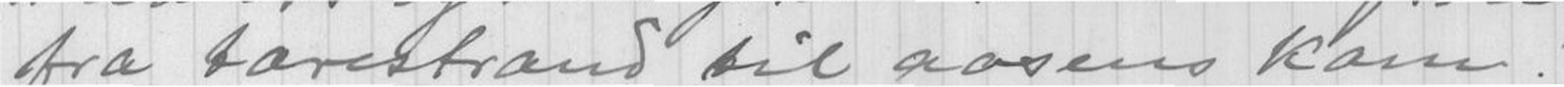fra tarestrand til osens kam!
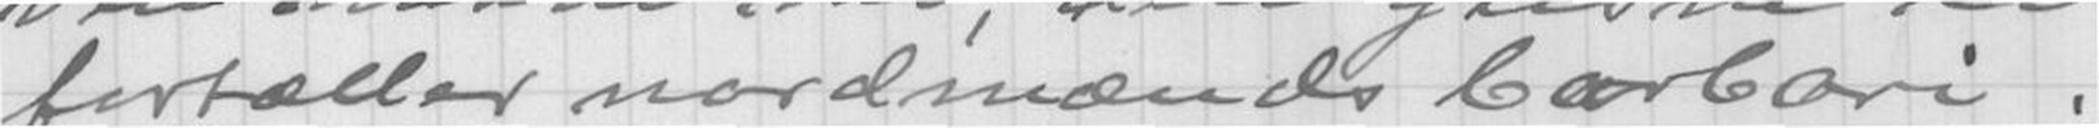fortæller nordmænds barbari.
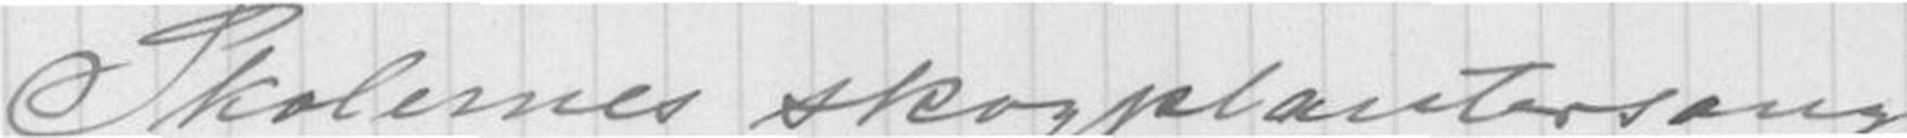Skolenes Skogplantesang
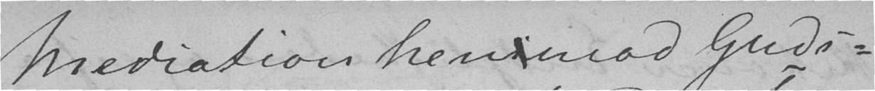Mediation henimod Guds-
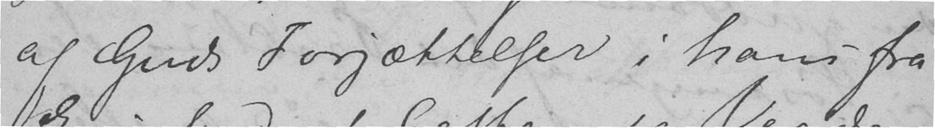af Guds Forjættelser i hans fra
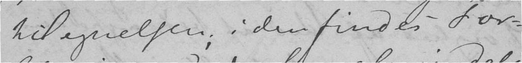tilegnelsen; i den findes For-
